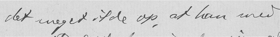det meget ilde op, at han med
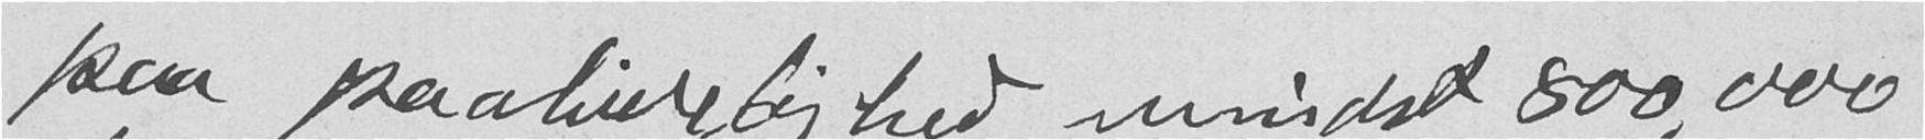paa paalidelighed mindst 800,000
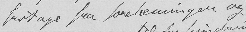fritage fra forelæsninger og
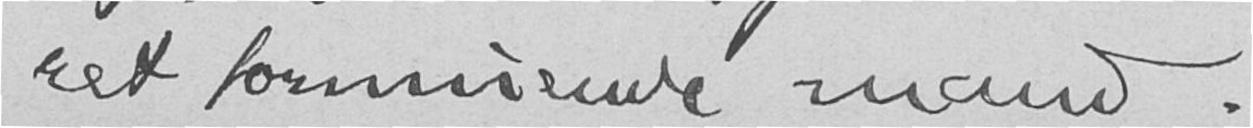ret formuende mand.
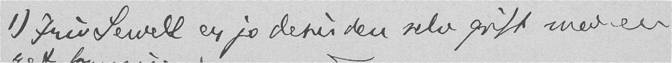1) Fru Sewell er jo desuden selv gift med en
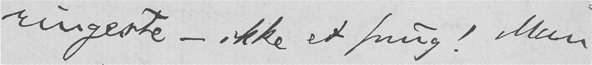ringeste - ikke et fnug! Man
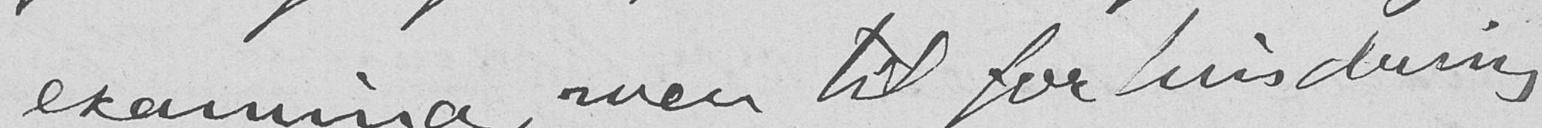examina, men til forhindring
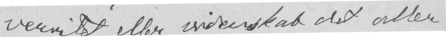versitet eller videnskab det aller-
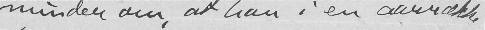minder om, at han i en aarrække
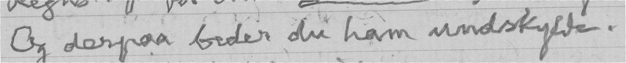Og derpaa beder du ham undskylde.
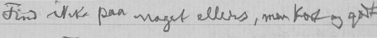Find ikke paa noget ellers, men kort og godt
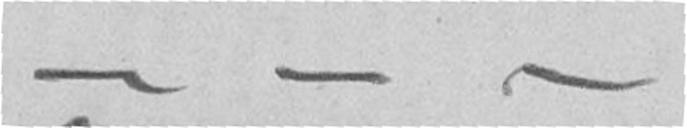- - -
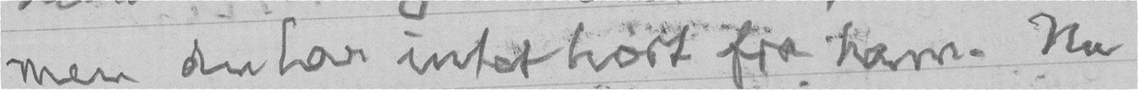men du har intet hørt fra ham. Nu
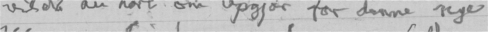vilde du høre om Opgjør for denne nye
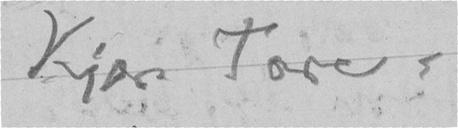Kjære Tore,
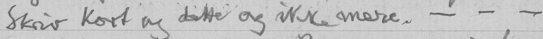Skriv kort og dette og ikke mere. - - -
